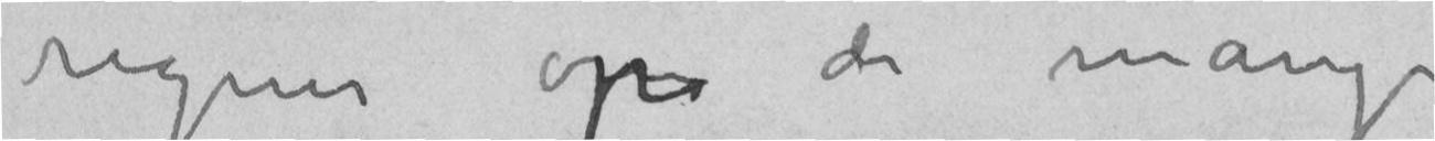regner op de mange
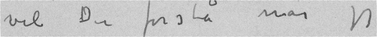vil Du forstå når jeg
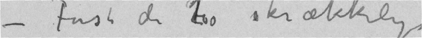– Først de to skrækkelige
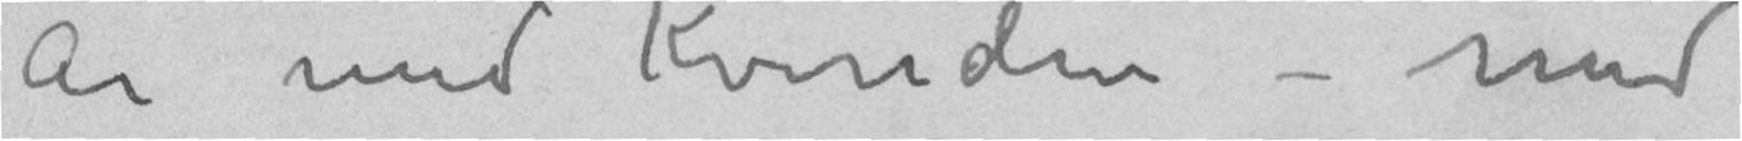år med Kvinden – med
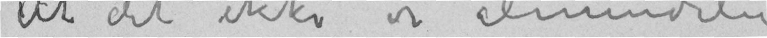At det ikke er almindelig
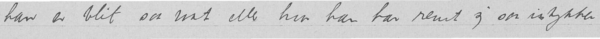han er blit saa vaat eller hvor han har revet sig saa istykker
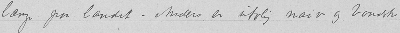længe paa landet. Anders er utrolig naiv og bondsk
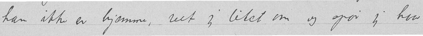han ikke er hjemme, vet jeg litet om og spør jeg hvor
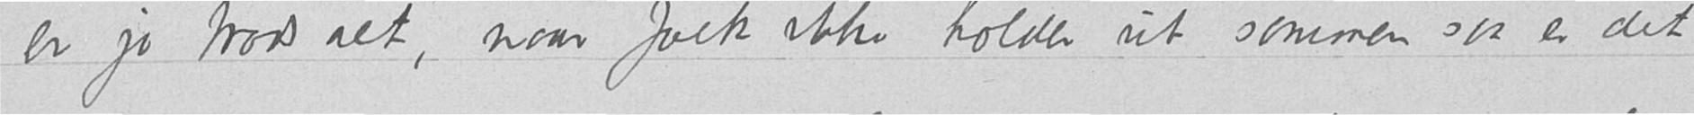er jo trods alt, naar folk ikke holder ut sammen saa er det
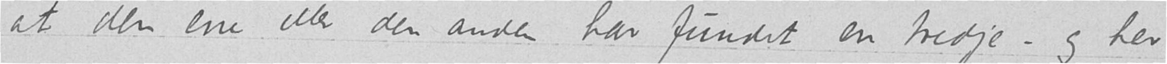at den ene eller den anden har fundet en tredje — og her
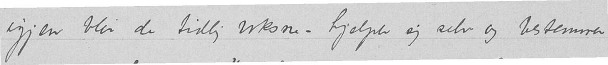igjen blir de tidlig voksne — hjelper sig selv og bestemmer
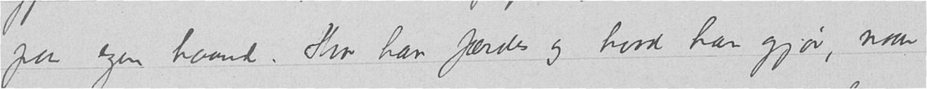paa egen haand. Hvor han færdes og hvad han gjør, naar
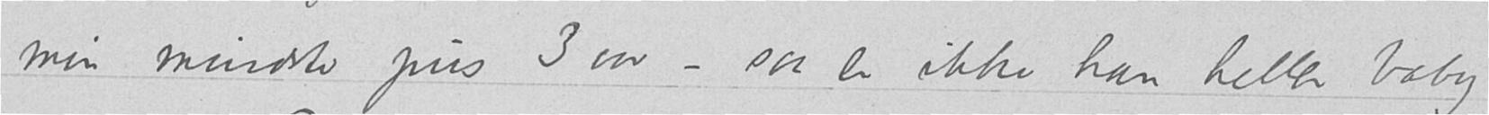min mindste pus 3 aar — saa er ikke han heller baby
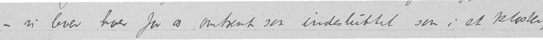— vi lever hver for os omtrent saa indesluttet som i et kloster,
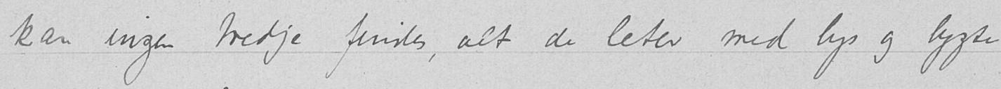kan ingen tredje findes, alt de leter med lys og lygte
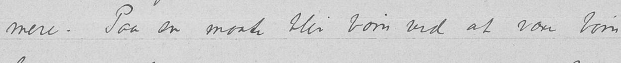mere. Paa en maate blir børn ved at være børn
